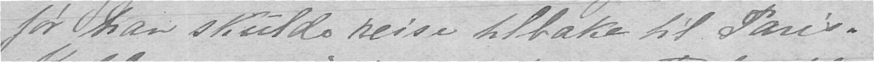før han skulde reise tilbake til Paris
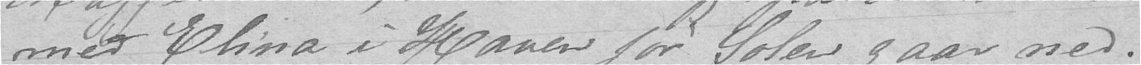med Elina i Haven for Solen gaar ned.
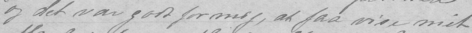og det var godt for mig, at faa vise mit
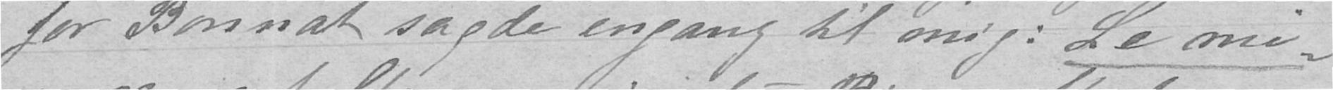for Bonnat sagde engang til mig: Le mi-
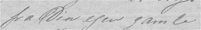fra Din egen gamle
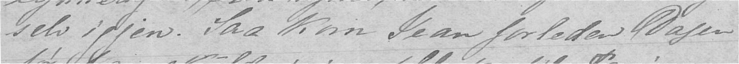selv igien. Saa kom Sean forleden Dagen
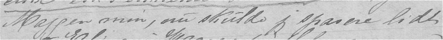Maggen min, nu skulde jeg spasere lidt
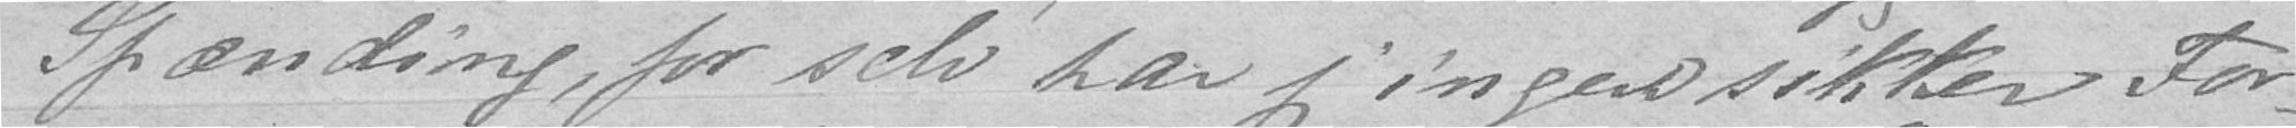Spænding, for selv har jeg ingen sikker For-
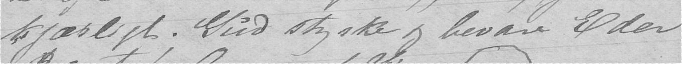kjærligt. Gud styrke og bevare Eder
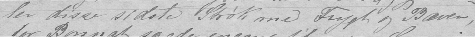ler disse sidste Strøk med Frygt og Bæven,
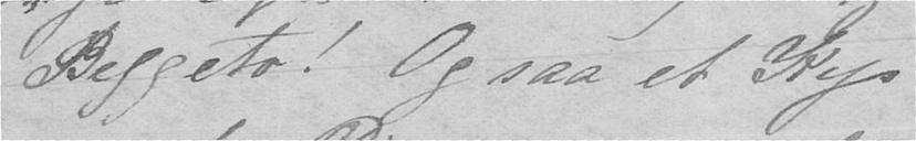Beggeto! Ogsaa et Kys
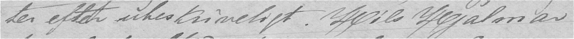ter efter ubeskriveligt. Hils Hjalmar
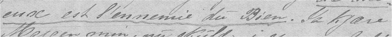eux est l'ennemie du Bien. Ja kjære
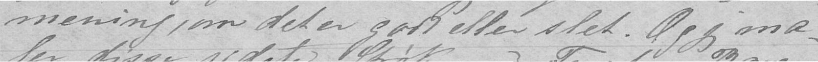mening, om det er godt eller slet. Og jeg ma-
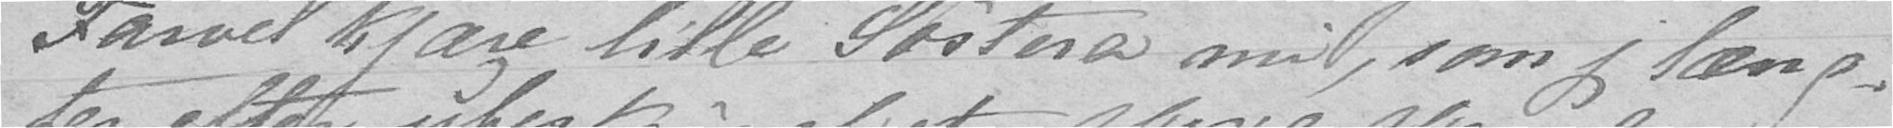Farvel Kjære lille Søstera mi, som jeg læng-
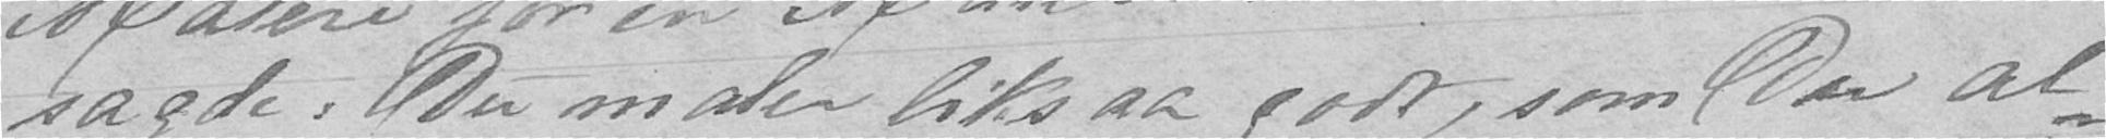sagde "Du maler liksaa godt, som Du al-
